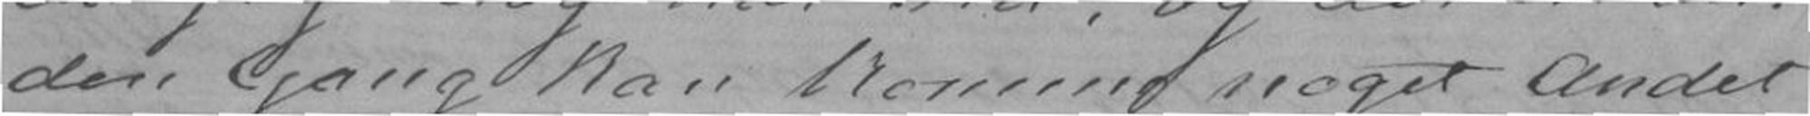den Gang kan komme noget Andet
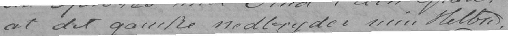at det ganske nedbryder min Helbred,
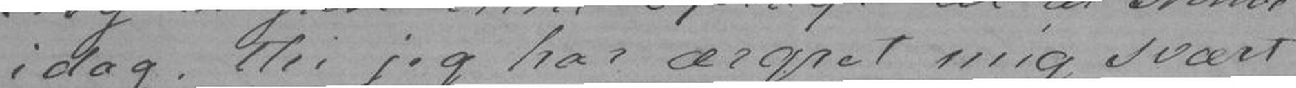idag, thi jeg ærgret mig svært
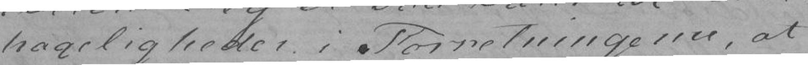hageligheder i Forretningerne, at
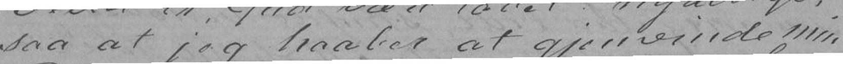saa at jeg haaber at gjenvinde min
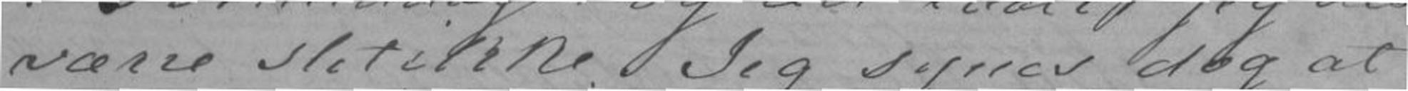værre slet ikke. Jeg synes dog at
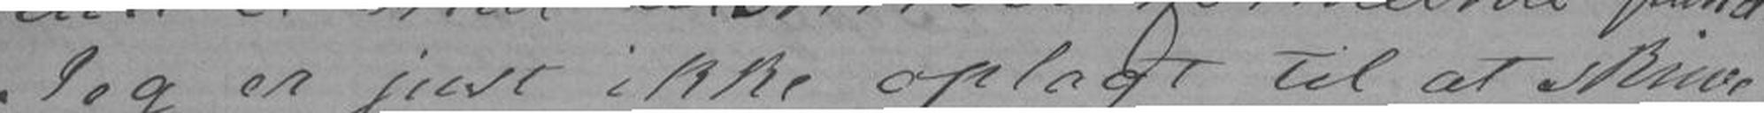Jeg er just ikke oplagt til at skrive
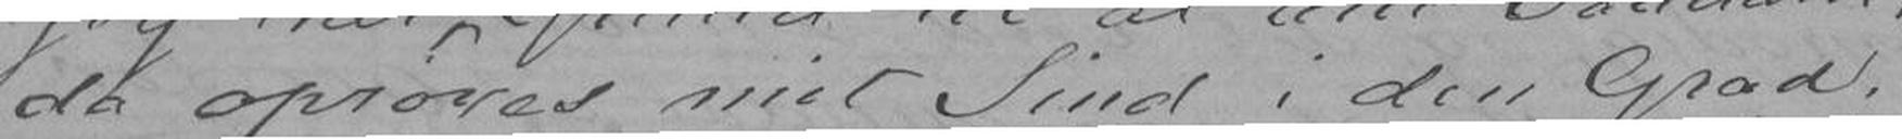da oprøres mit Sind i den Grad,
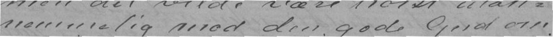nemmelig mod den gode Gud om
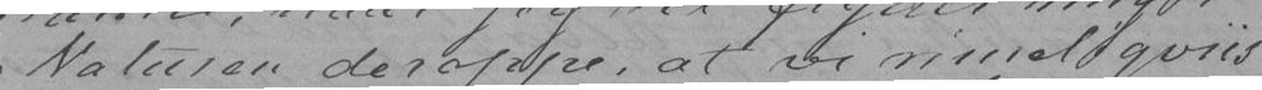Naturen deroppe, at vi rimeligviis
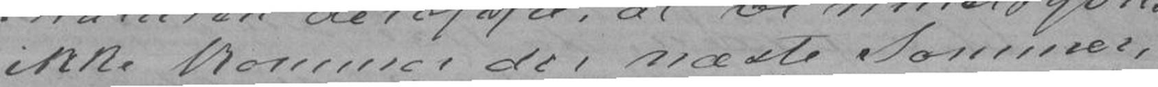ikke kommer der næste Sommer,
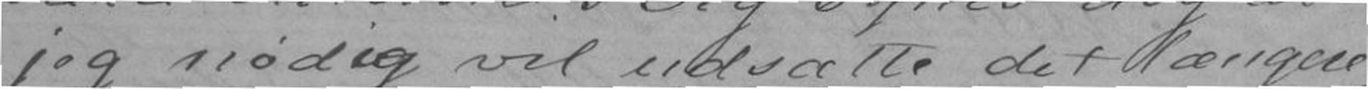jeg nødig vil udsætte det længere,
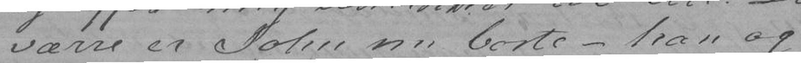værre er John nu borte - han og
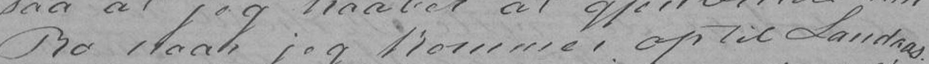Ro naar jeg kommer op til Landaas.
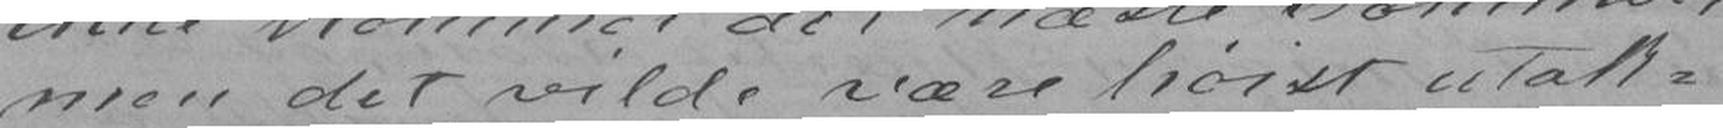men det vilde være høist utak-
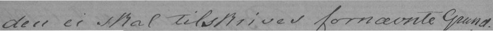den ei skal tilskrives fornævnte Grund.
